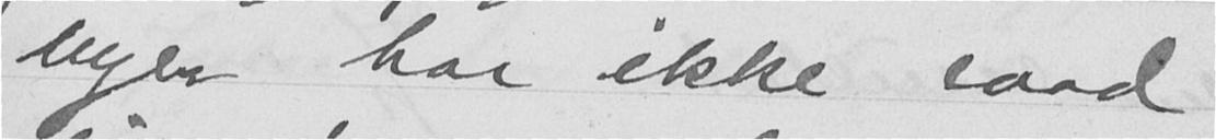byer har ikke raad
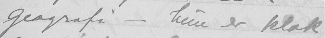geografi - hun er klok
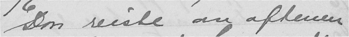Don reiste om aftenen
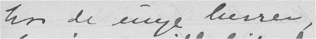hos de unge herrer,
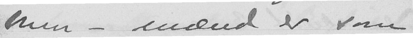Men - mænd er som
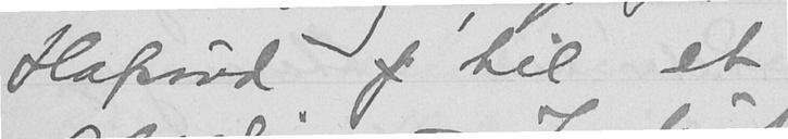Hafsrød p til et
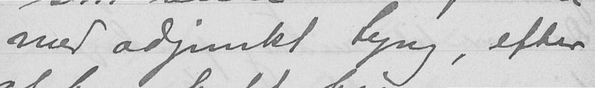med adjunkt Lyng, efter
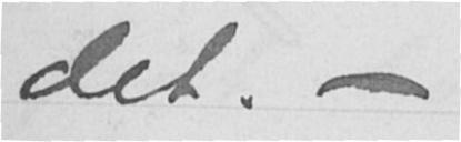det. -
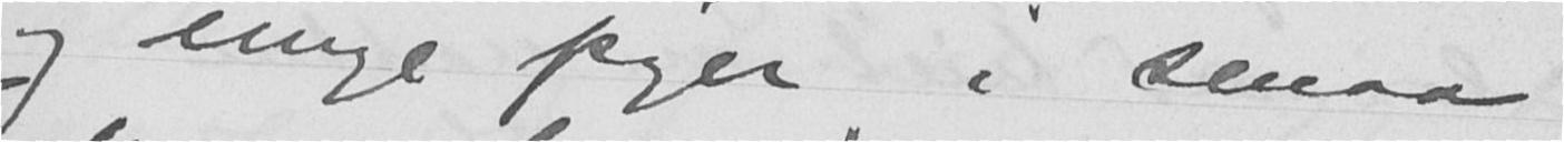og unge piger i smaa
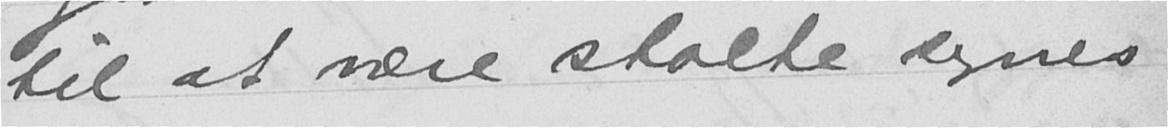til at være stolte synes
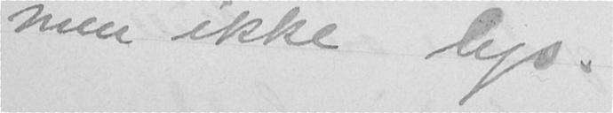men ikke lys.
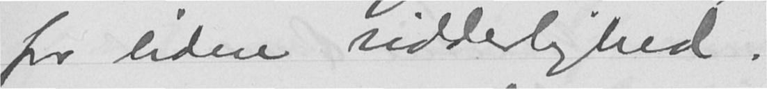for liden ridderlighed.
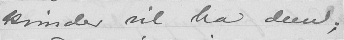kvinder vil ha dem;
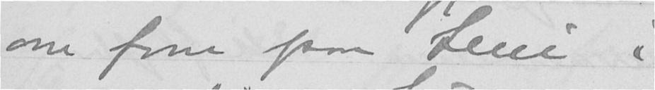om form paa Leni i
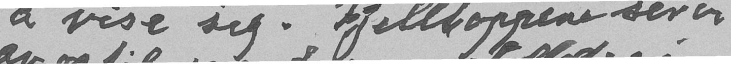å vise seg. Fjelltoppene ser vi
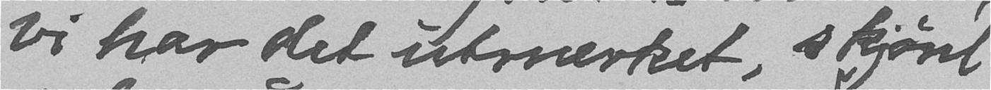vi har det utmerket, skjønt
